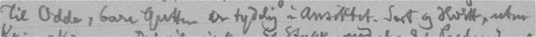til Odde, bare Gutten er tydelig i Ansiktet. Sort og Hvitt, uten
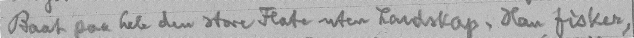Baat paa hele den store Flate uten Landskap. Han fisker,
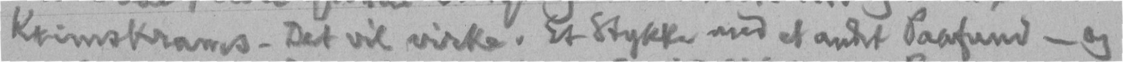Krimskrams. Det vil virke. Et Stykke med et andet Paafund - og
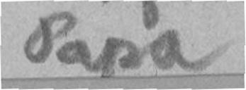Papa
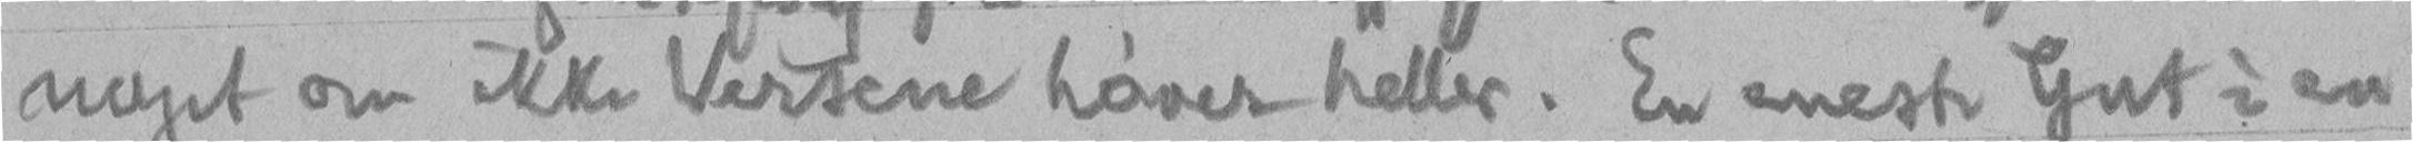noget om ikke Versene høver heller. En eneste Gut i en
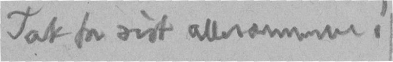Tak for sist allesammen!
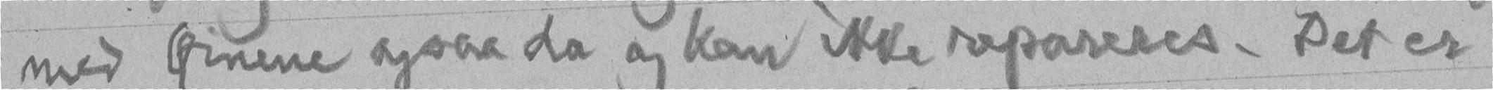med Øinene ogsaa da og kan ikke repareres. Det er
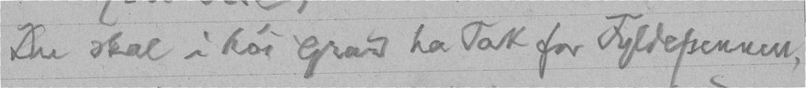Du skal i høi Grad ha Tak for Fyldepennen,
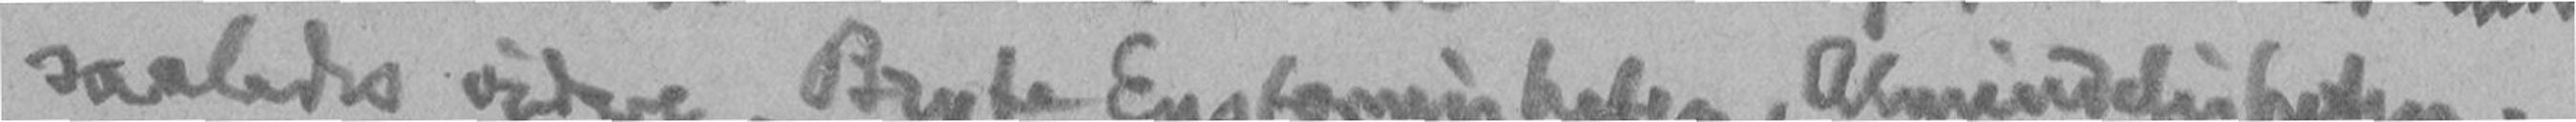saaledes videre. Bryte Ensformigheten, Almindeligheten.
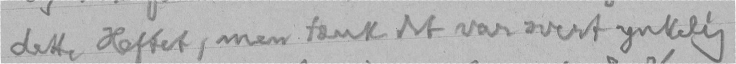dette Heftet, men tænk det var svert ynkelig
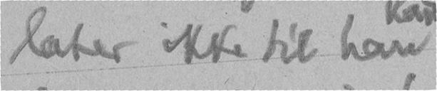later ikke til han
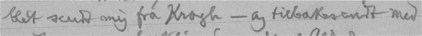blit sendt mig fra Krogh - og tilbakesendt med
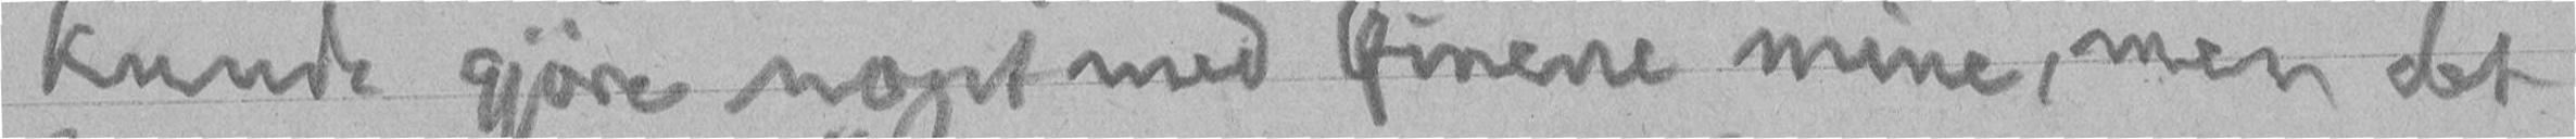kunde gjøre noget med Øinene mine, men det
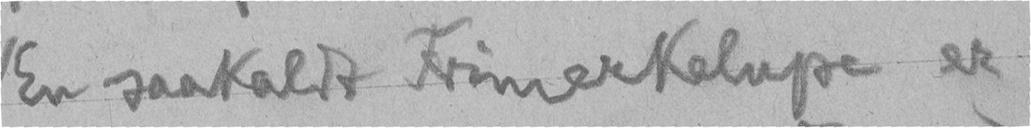En saakaldt Frimerkelupe er
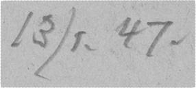13/1. 47.
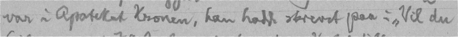var i Apoteket Kronen, han hadde skrevet paa: "Vil du
